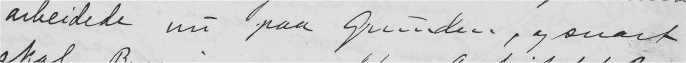arbeidede nu paa Grunden, og snart
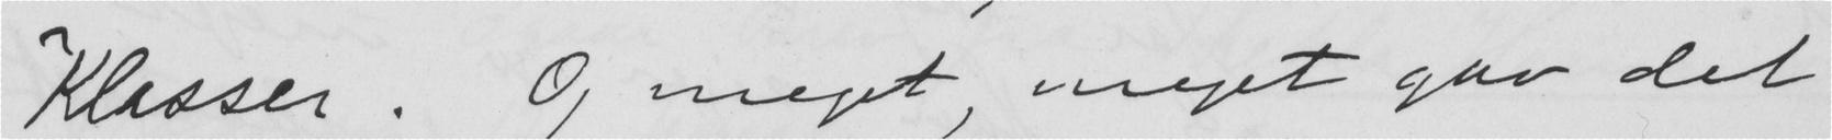Klasser. Og meget, meget gav det
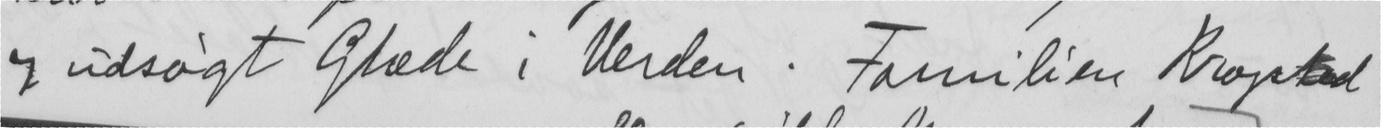og udsøgt Glæde i Verden. Familien Krogstad
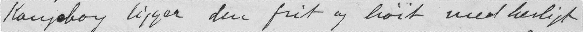Kongsborg ligger den frit og høit med herligt
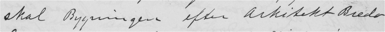skal Bygningen efter Arkitekt Brede
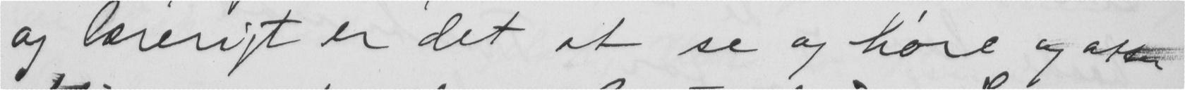og lærerigt er det at se og høre og atter
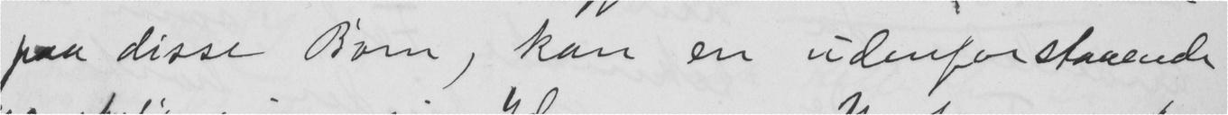paa disse Børn, kan en udenforstaaende
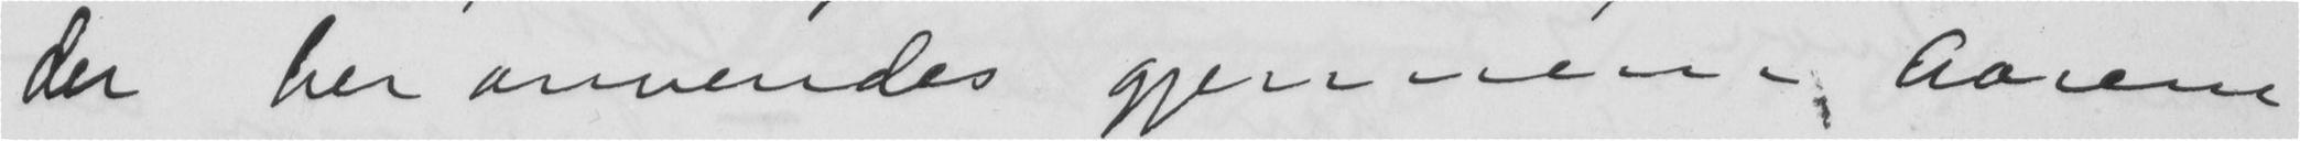der her anvendes gjennem i Aarene
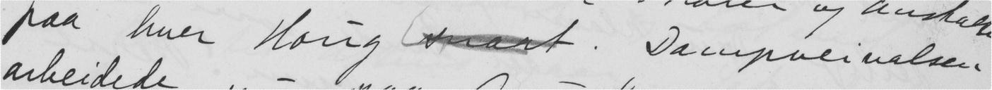paa hver Haug . Dampveivalsen
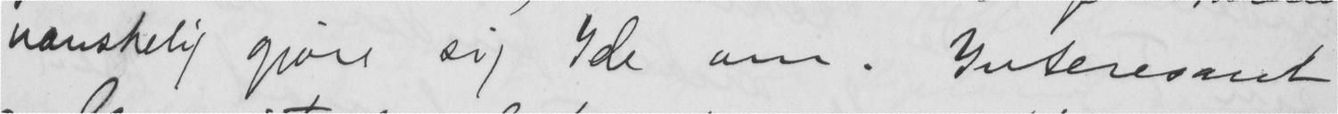vanskelig gjøre sig Ide om. Interesant
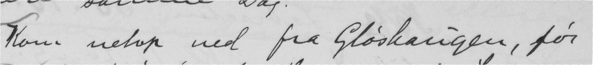Kom netop ned fra Gløshaugen, før
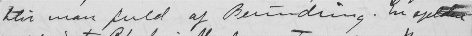blir man fuld af Beundring. En sjelden
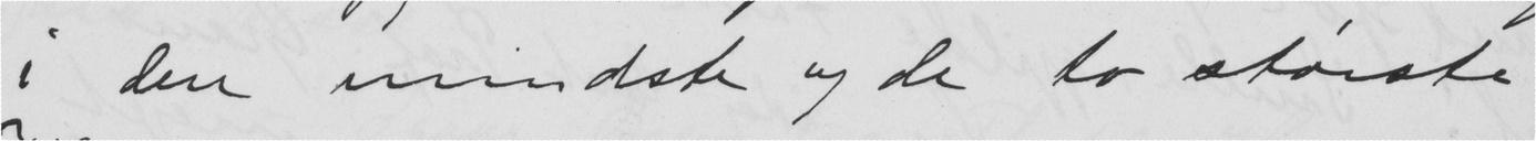i den mindste og de to største
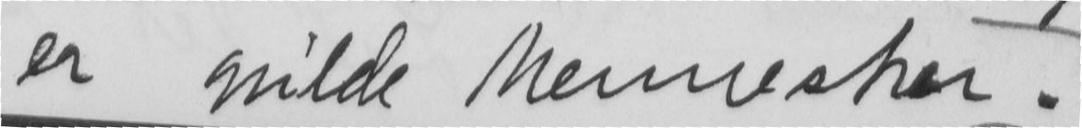er milde Mennesker -
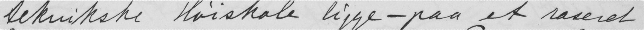teknikske Høiskole ligge - paa et raseret
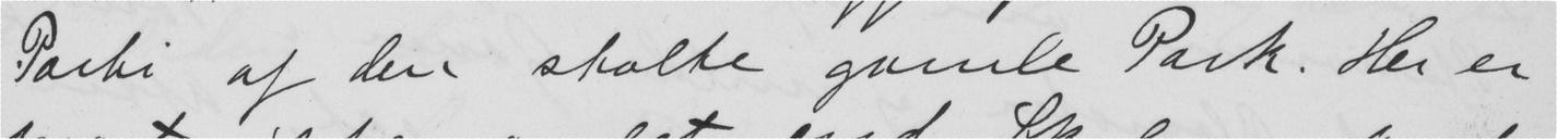Parti af den stolte gamle Park. Her er
